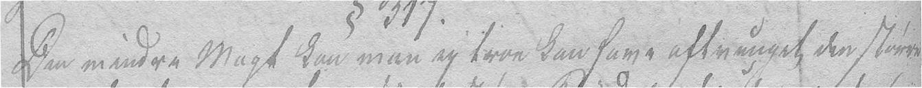Den mindre Magt kan man ey troe kan have aftvunget den større
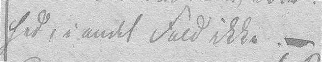hed, i andet Fald ikke. –
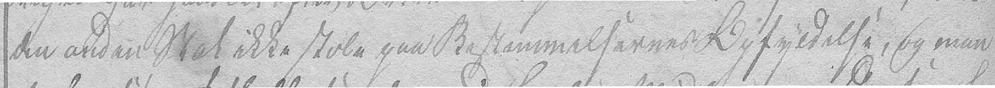den anden Stat ikke stole paa Bestemmelsernes Opfyldelse, og maa
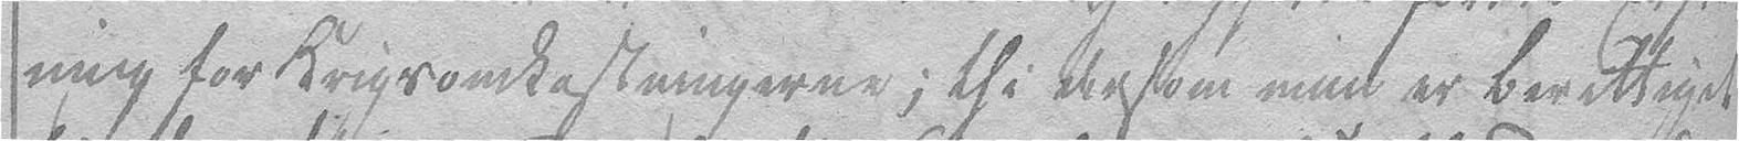ning for Krigsomkostningerne; thi dersom man er berettiget
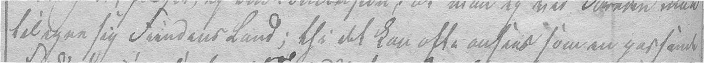tilegne sig Fiendens Land; thi det kan ofte ansees som en passende
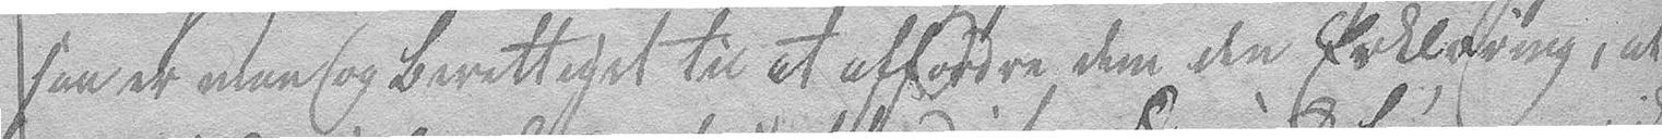saa er man og berettiget til at affordre dem den Erklæring, at
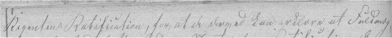Regentens Ratification, for at de derved kan erklære at Fuldmæg-
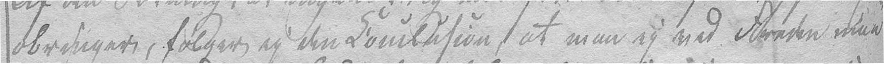obringer, følger ey den Conclusion, at man ey ved Freden maa
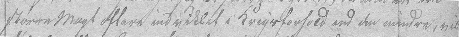større Magt oftere indviklet i Krigsforhold end den mindre, vil
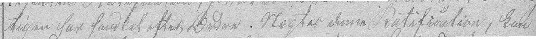tigen har handlet efter Ordre. Nægtes denne Ratification, kan
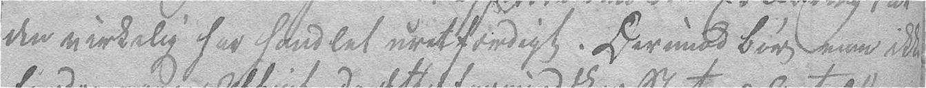den virkelig har handlet uretfærdigt. Derimod bør man ikke
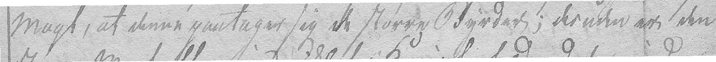Magt, at denne paatager sig de større Byrder; desuden er den
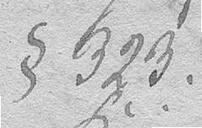§ 323.
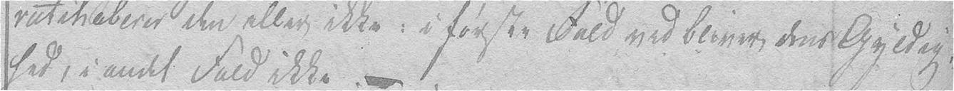ratihaberer den eller ikke: i første Fald vedbliver dens Gyldig-
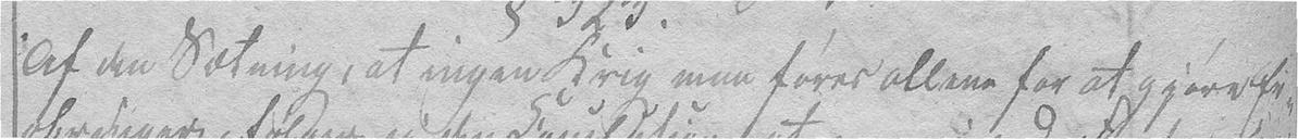Af den Sætning, at ingen Krig maa føres allene for at gjøre Er-
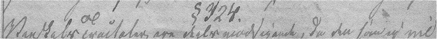Venskabs Tractater ere deels modsigende, da den som ey vil
